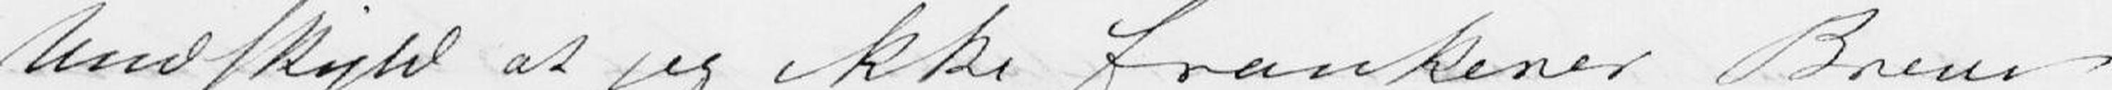Undskyld at jeg ikke frankerer Breve
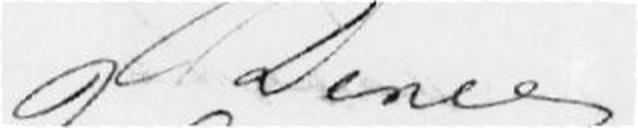Deres
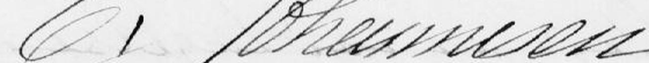C. Johannesen
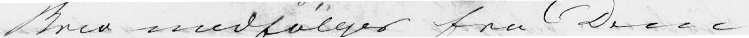Brev medfølger fra Dem
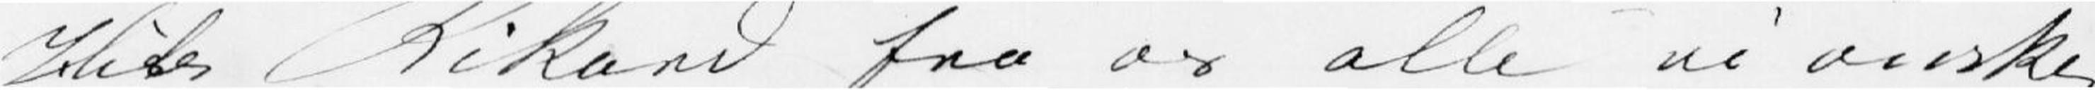Hils Rikard fra os alle vi ønsker
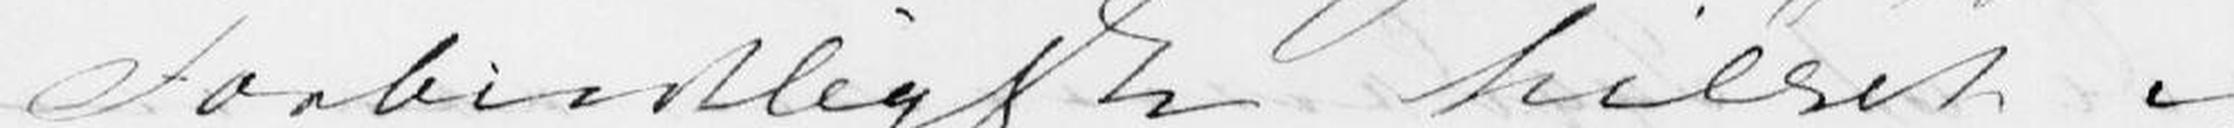Forbindeligte hilset.
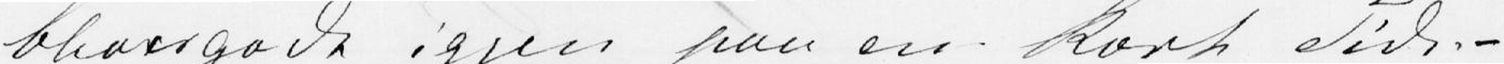bliver godt igjen paa en kort Tid. -
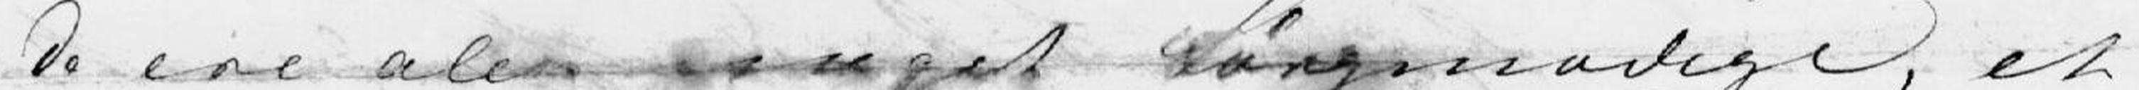De ere alle meget Sørgmodige, et
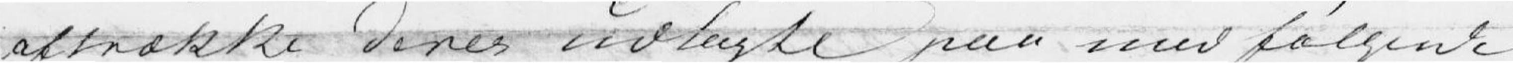aftrække Deres udbyte paa med følgende
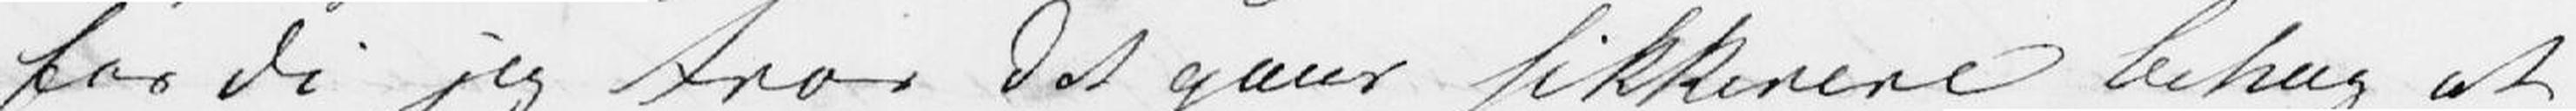fordi jeg tror Det gaar sikkerere behag at
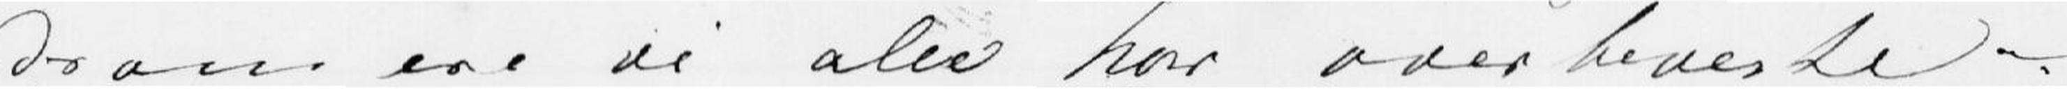derom ere vi alle her overbeviste;
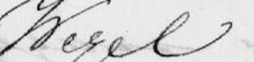Wexel
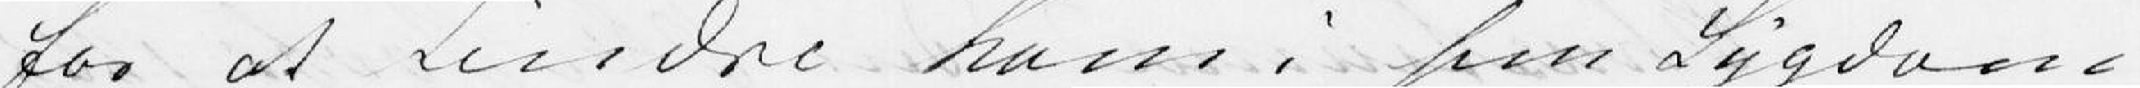for at Lindre ham i sin Sygdom
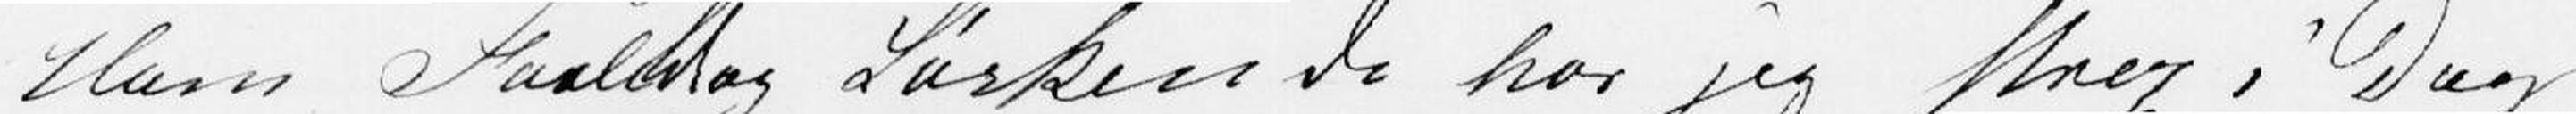Ham Faaltdag Søskende har jeg strax i Dag
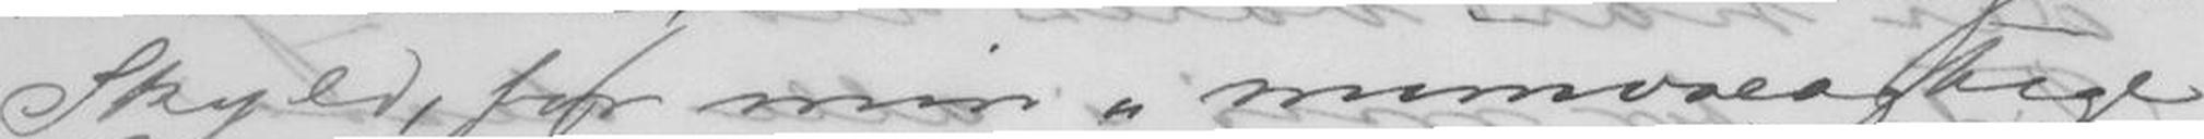Skyld, for min mimoseagtige
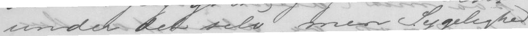under det selv, men Sygelighed
